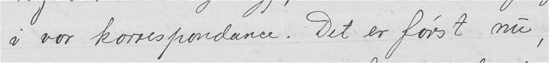i vor korrespondance. Det er først nu,
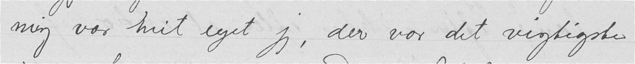meg var mit eget jeg, der var det vigtigste
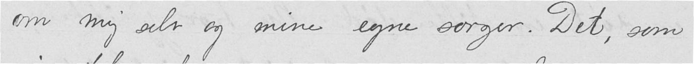om mig selv og mine egne sorger. Det, som
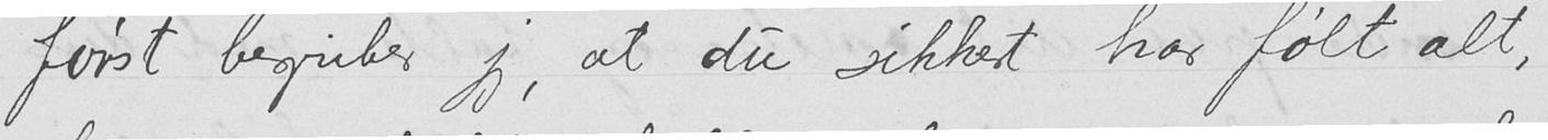først begriber jeg, at du sikkert har følt alt,
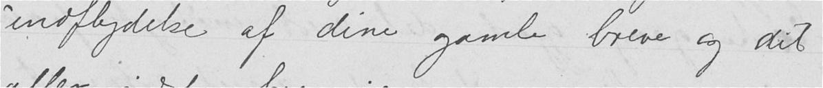indflydelse af dine gamle breve og det
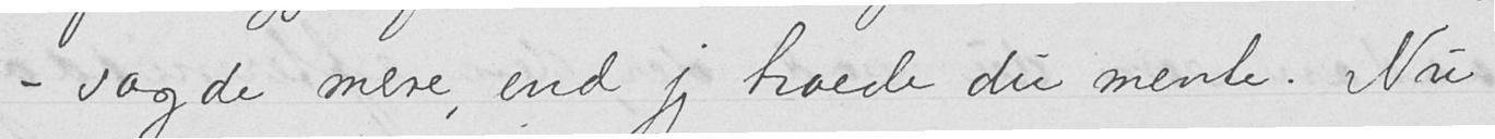- sagde mere, end jeg troede du mente. Nu
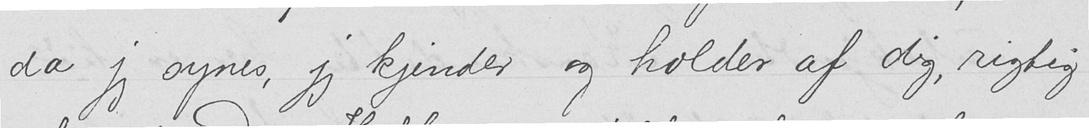da jeg synes, jeg kjender og holder af dig, rigtig
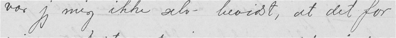var jeg mig ikke selv bevidst, at det for
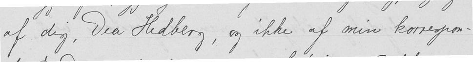af dig, Dea Hedberg, og ikke af min korrespon-
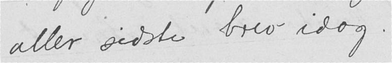aller sidste brev idag.
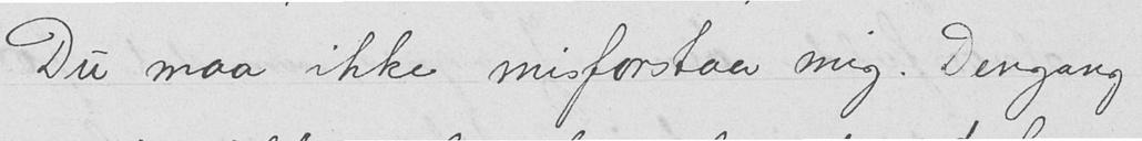Du maa ikke misforstaa mig. Dengang
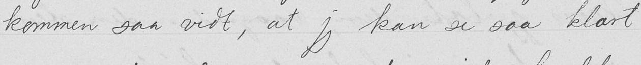kommen saa vidt, at jeg kan se saa klart
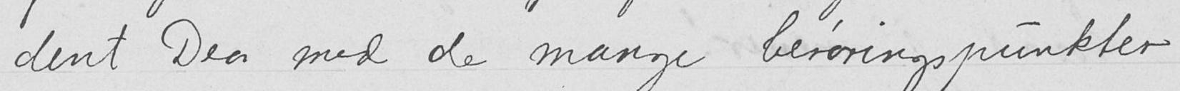dent Dea med de mange berøringspunkter
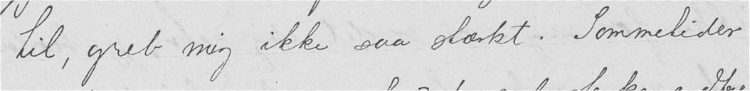til, greb mig ikke saa stærkt. Sommetider
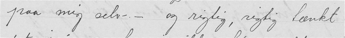paa mig selv - og rigtig, rigtig tænkt
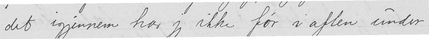det igjennem har jeg ikke før i aften under
